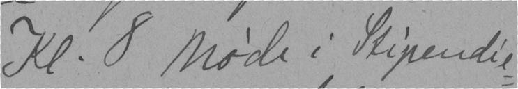Kl. 8 Møde i Stipendie-
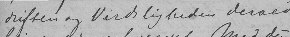driften og Verdsligheden derved
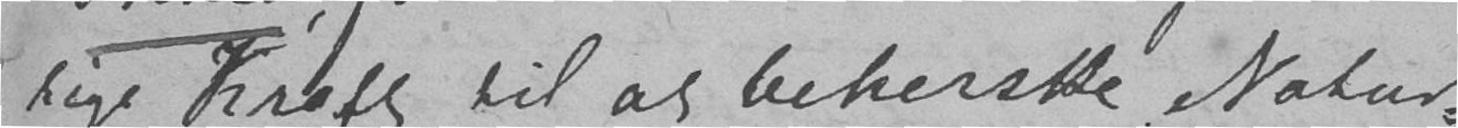lige Kraft til at beherske Natur-
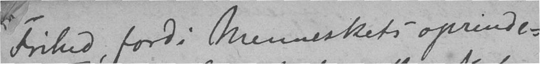Frihed, fordi Menneskets oprinde-
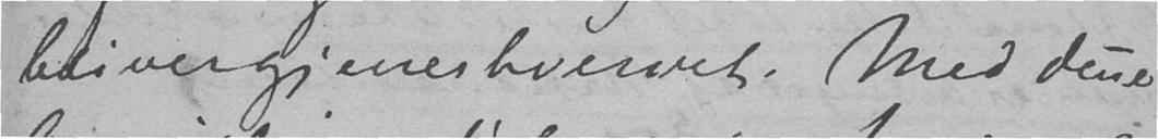bliver gjenerhvervet. Med denne
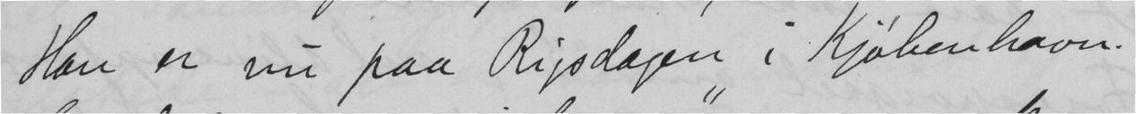Han er nu paa Rigsdagen i Kjøbenhavn.
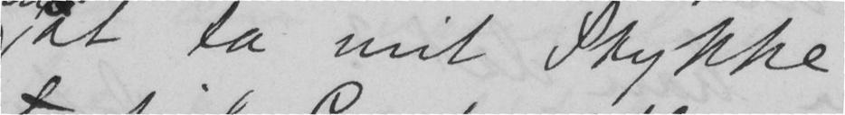at ta mit Stykke
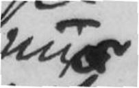nu
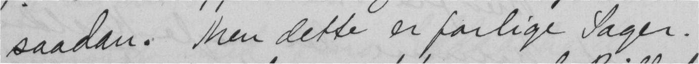saadan. Men dette er forlige Sager.
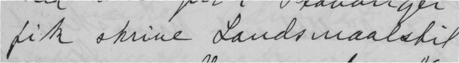fik skrive Landsmaalstil
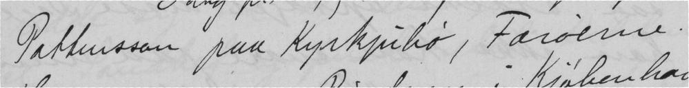Pattursson paa Kyrkjubø, Færøerne.
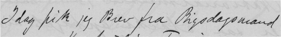Idag fik jeg Brev fra Rigsdagsmand
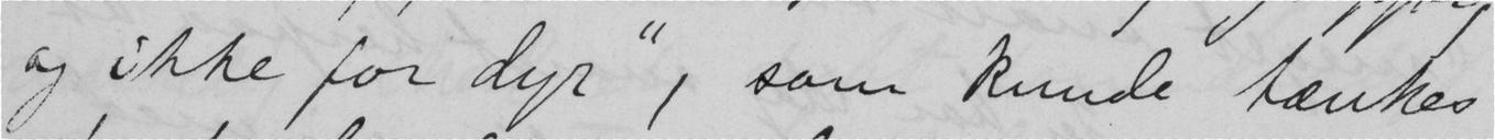og ikke for dyr", som kunde tænkes
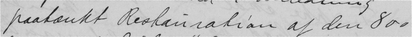paatænkt Restauration af den 800
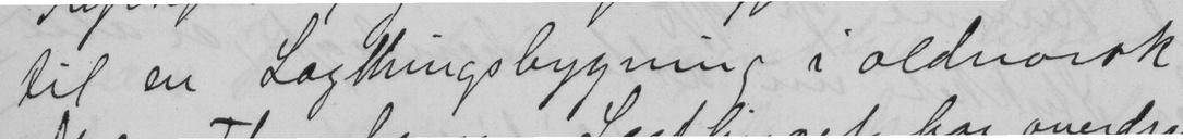til en Lagthingsbygning i oldnorsk
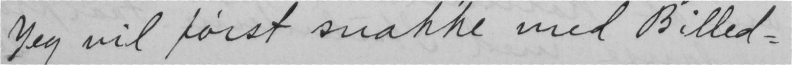Jeg vil først snakke med Billed-
